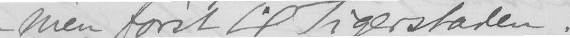men først til Tigerstaden.
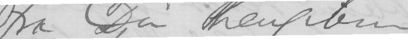fra Din hengivne
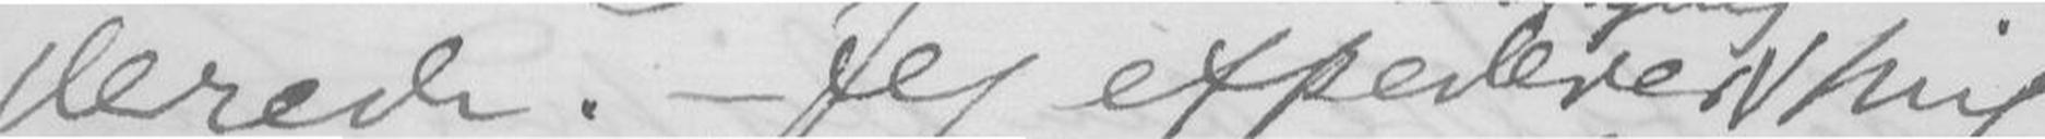derede. Jeg expederer mig
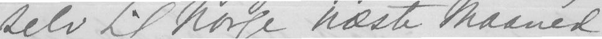selv til Norge næste Maanded
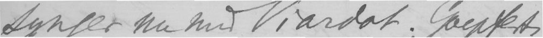synger nu med Viardot. Geepfert
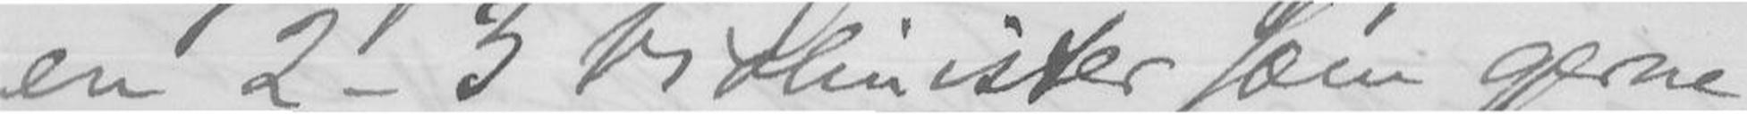en 2-3 Violinister som gærne
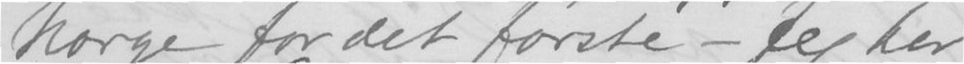Norge for det første - Jeg har
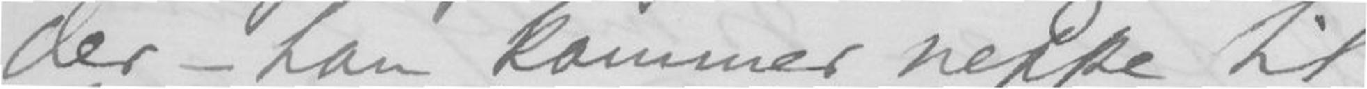der - han kommer neppe til
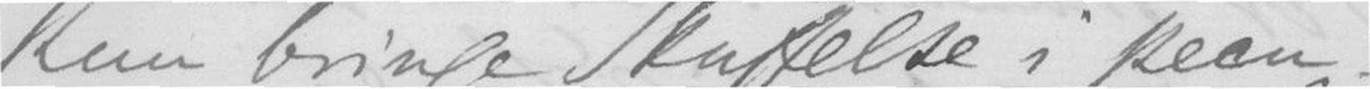kun bringe Skuffelse i pee-
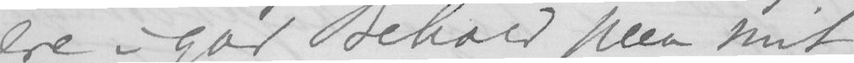ere i god Behold paa mit
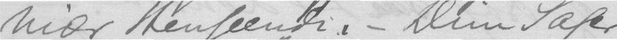niær Henseende. - Dine Sager
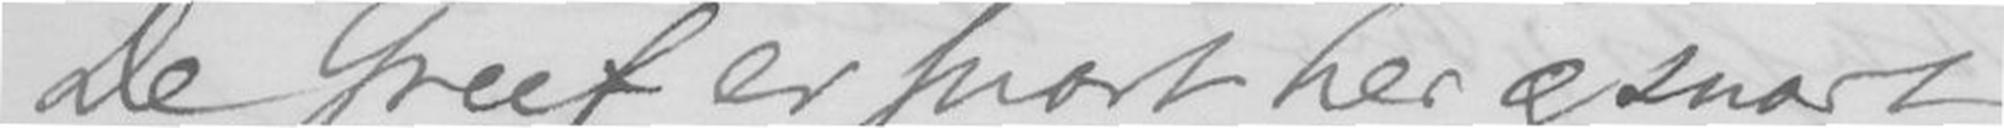De Greef er snart her og snart
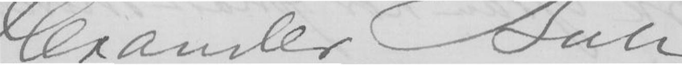Alexander Bull
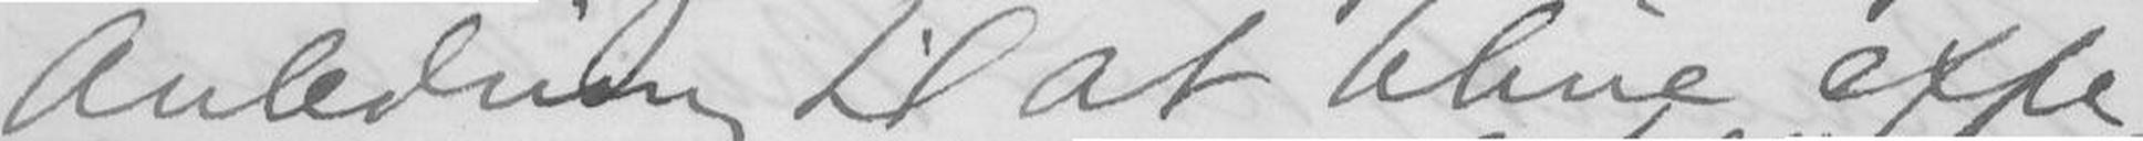Anledning til at blive efpe-
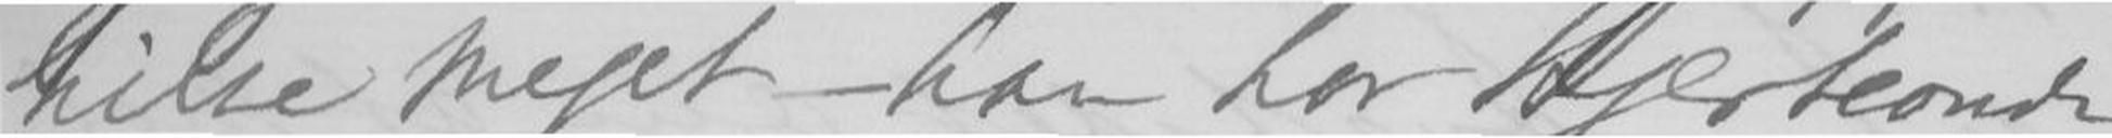hilse meget han har Hjerteonde
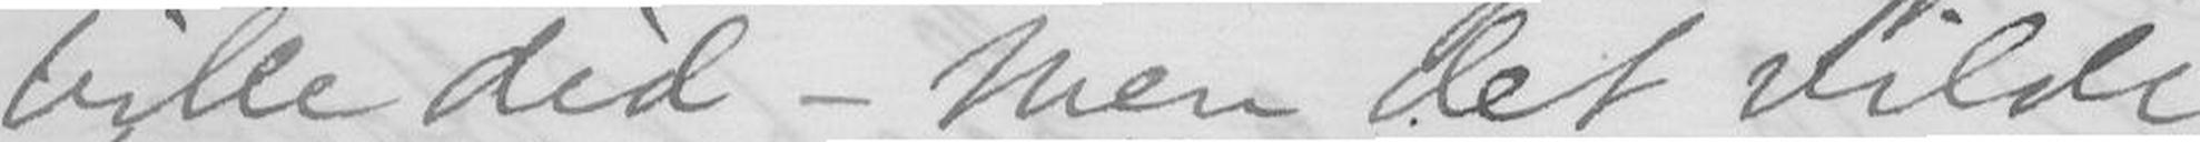ville did. Men det vilde
